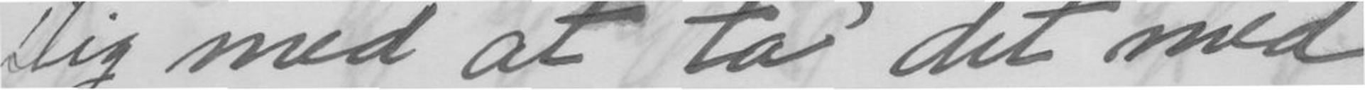Dig med at ta' det med
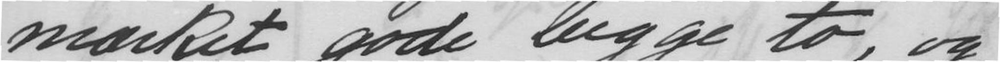mærket gode begge to, og
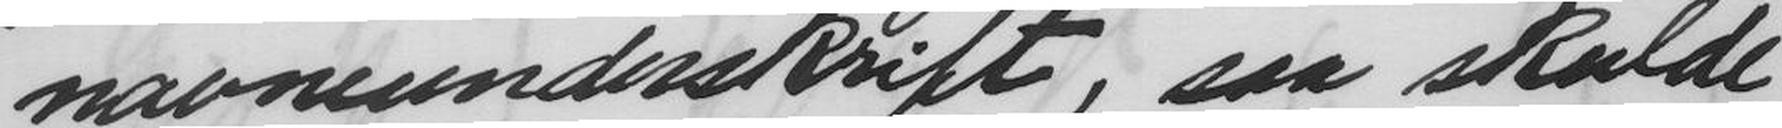navneunderskrift, saa skulde
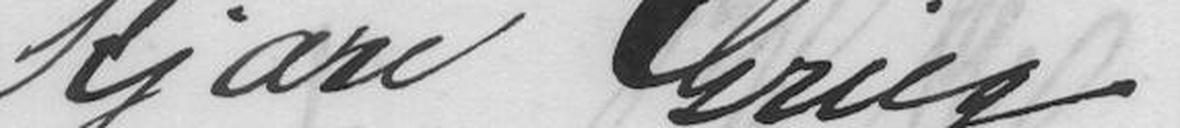Kjære Grieg
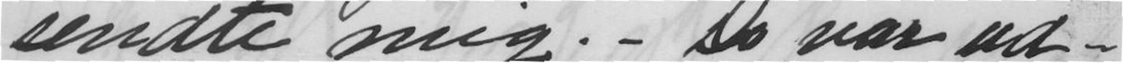sendte mig. - De var ud-
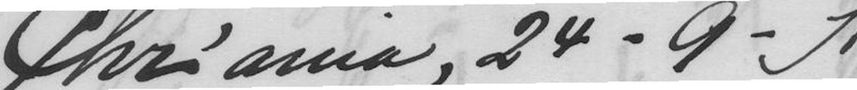Chr'ania, 24-9-1898.
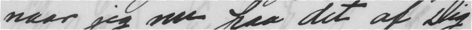naar jeg nu paa det af Dig
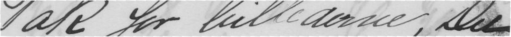Tak for billederne, Du
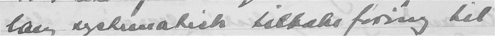lang systematisk tilbakeføring til
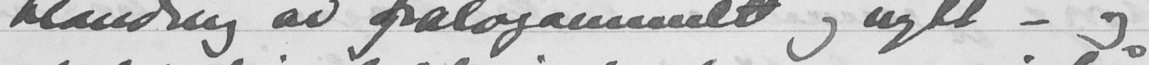blanding av halvgammelt og nytt - og
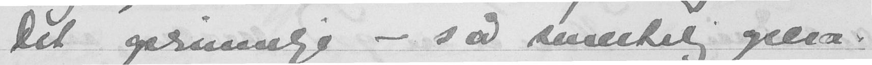det opprinnelige - så smertelig gelsa!
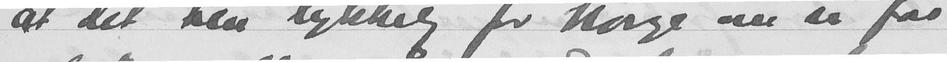at det blir lykkelig for Norge om vi får
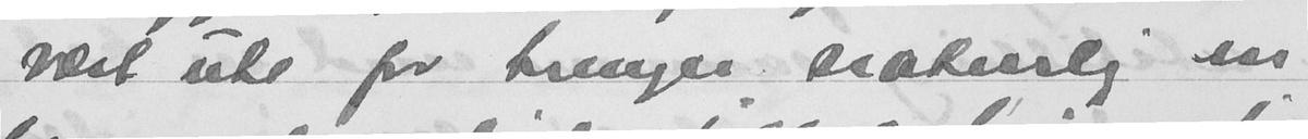vært ute for trenger virkelig en
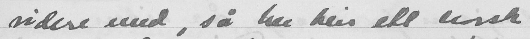videre med, så her blir ett norsk
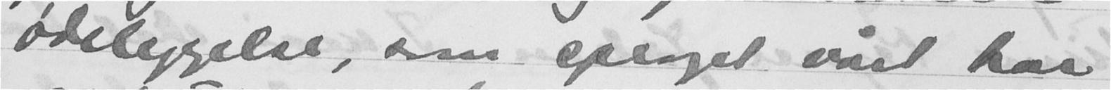ødelggelse, som sproget vårt har
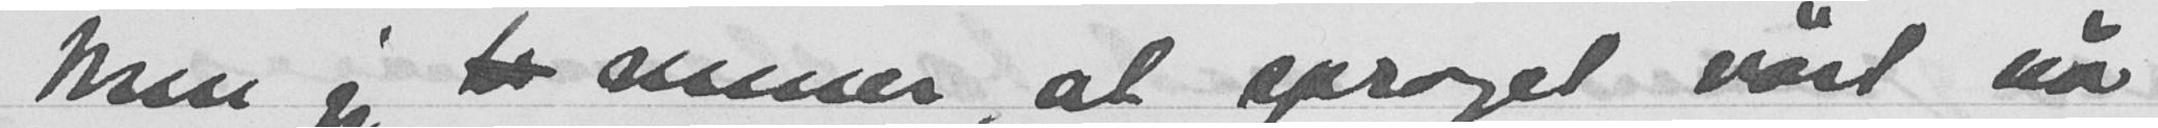Men jeg mener, at sproget vårt nå
